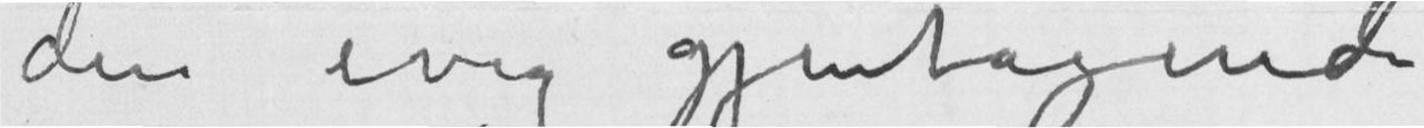den evig gjentagende
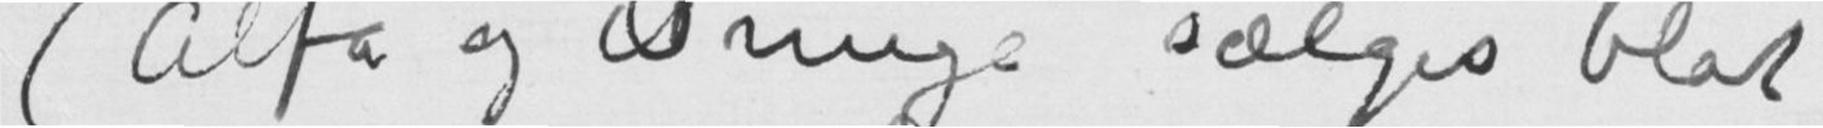(Alfa og Omega sælges blot
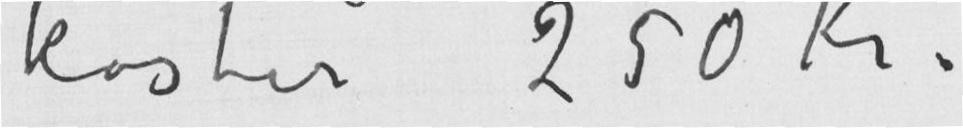koster 250 Kr.
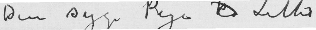Den syge Pige k Litho
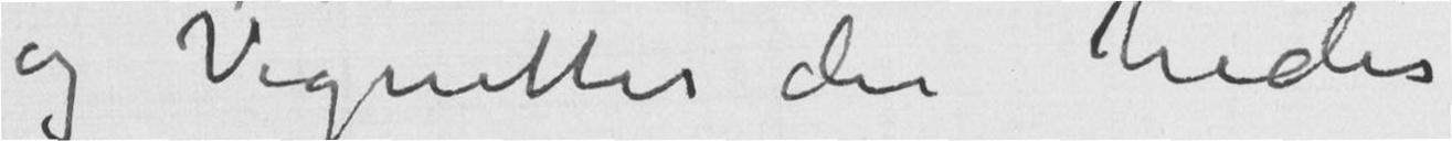og Vignetter der heder
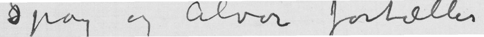Spøg og Alvor fortæller
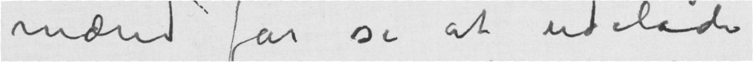mænd får se at udelade
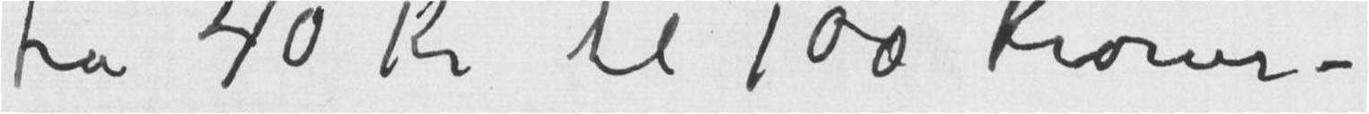for 40 Kr til 100 Kroner –
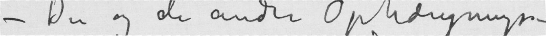– Du og de andre Ophængnings-
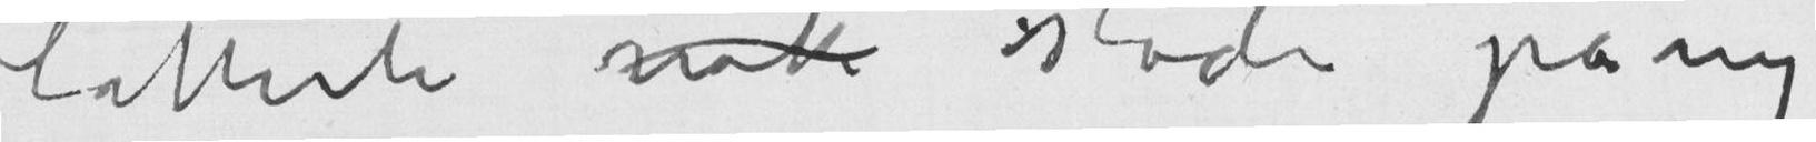latterli nok stadi på ny
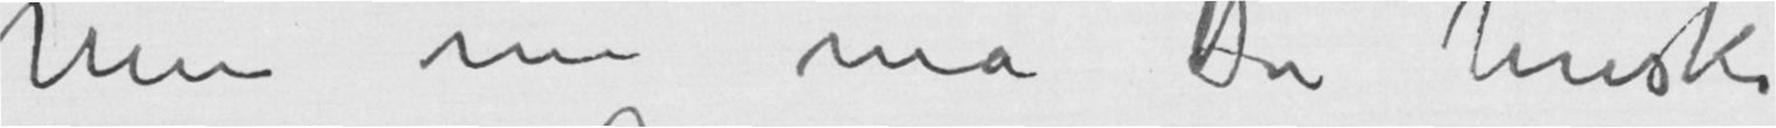Men nu må Du huske
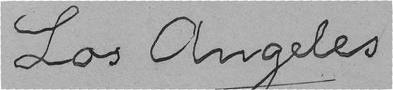Los Angeles
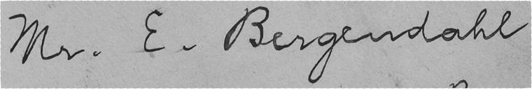Mr. E. Bergendahl
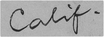Calif.
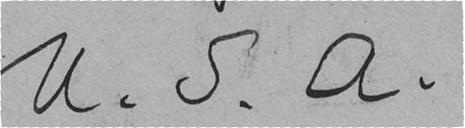U. S. A.
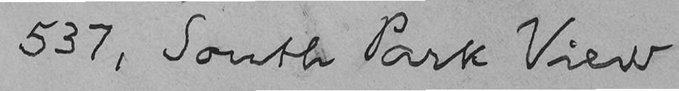537, South Park View
